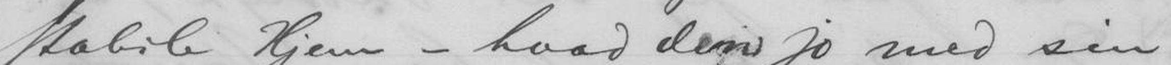stabile Hjem - hvad den jo med sin
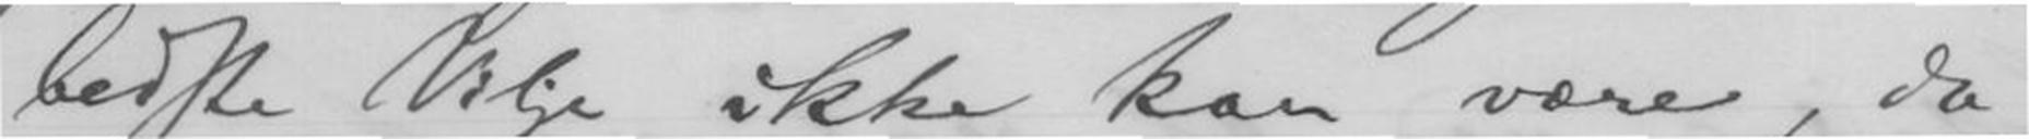bedste Vilje ikke kan være, da I
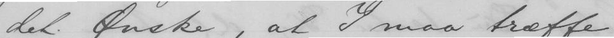det Ønske, at I maa træffe
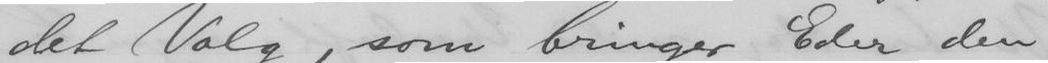det Valg, som bringer Eder den
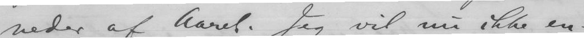neder af Aaret. Jeg vil nu ikke en-
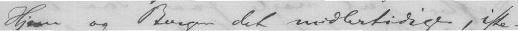Hjem og Bergen det midlertidige, iste-
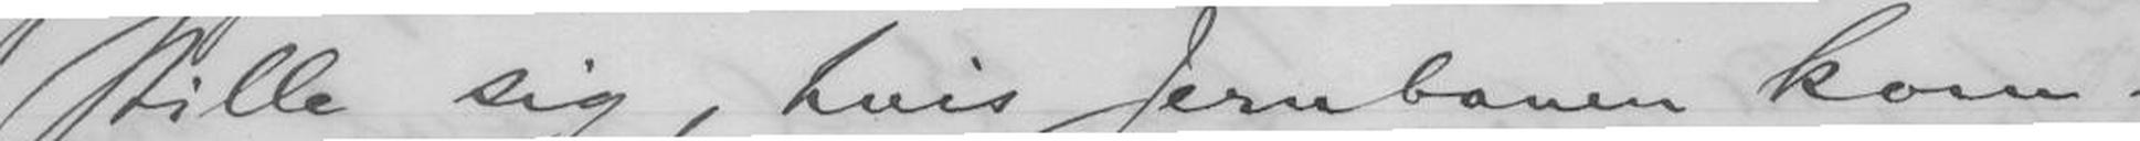stille sig, hvis Jernbanen kom-
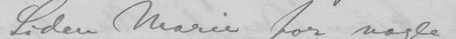Siden Marie for nogle Dage
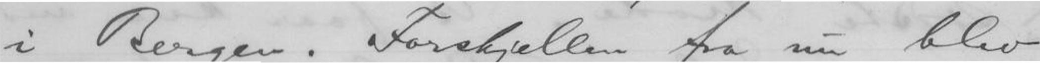i Bergen. Forskjellen fra nu blev
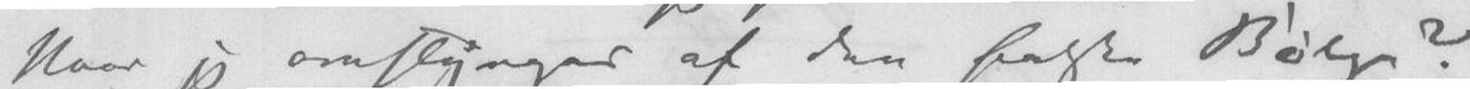Hvor jeg omslynges af den fagre Bølge?
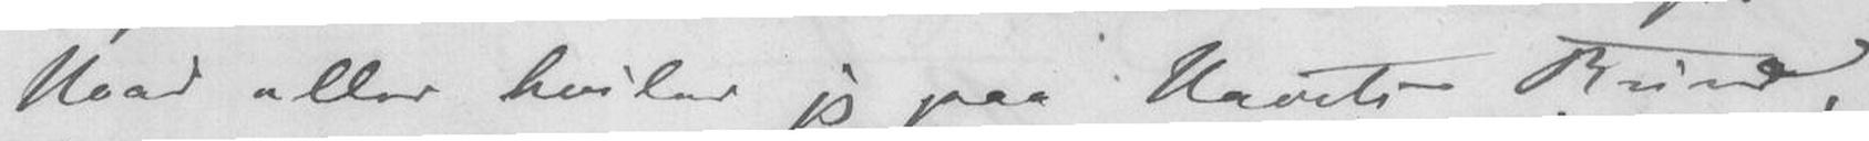Hvad eller hviler jeg paa Havets Bund,
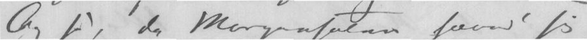Og se, da Morgensolen favn sig,
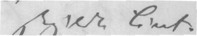skyldte Livet.
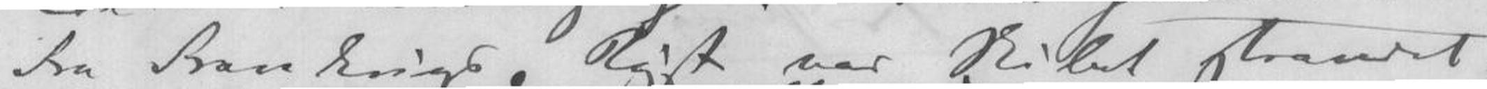Fra Frankrige Kyst var Skibet strandet;
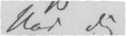Hos dig
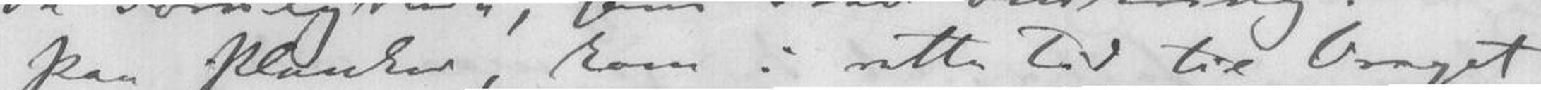Paa Planker, kom i rette Tid til Vraget
